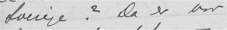Sverige? Da er vor
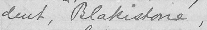dent, Blakistone,
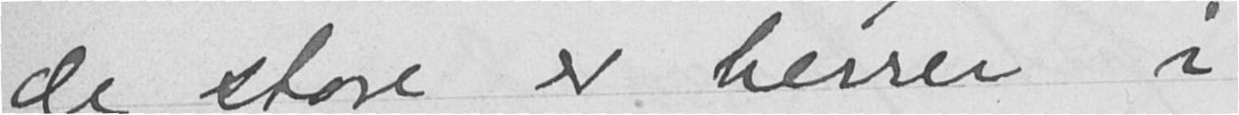de store er herrer i
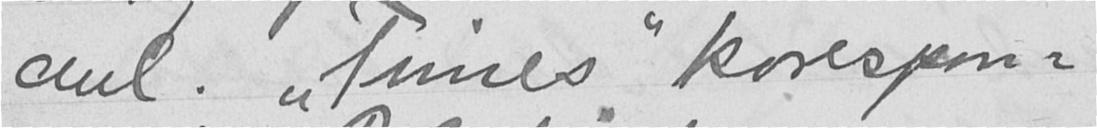anl. "Times" korespon-
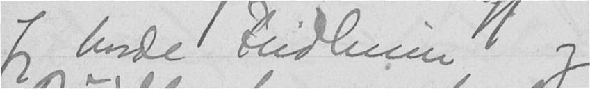Jeg havde Fridheim og
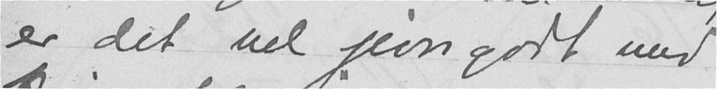er det vel jevngodt med
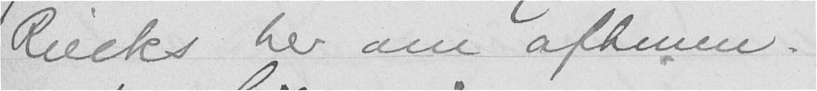Riecks her om aftenen.
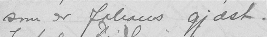som er Johans gjæst.
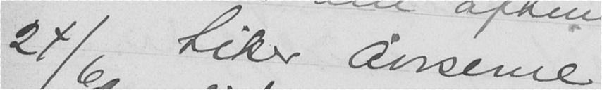24/6 Liker aviserne
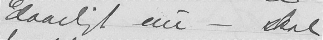daarligt nu - skal
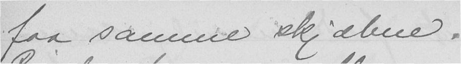faa samme skjæbne.
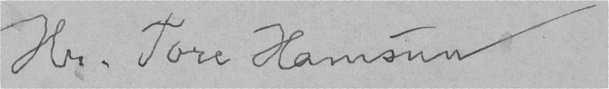Hr. Tore Hamsun
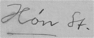Høn St.
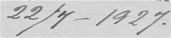22/7 - 1927.
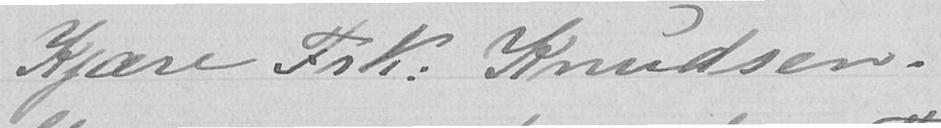Kjære Frk. Knudsen.
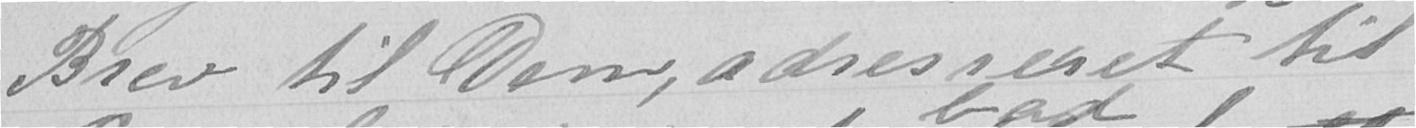Brev til Dem, adresseret til
